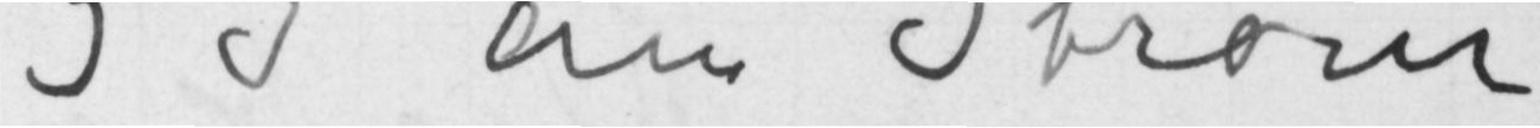53 am Strom
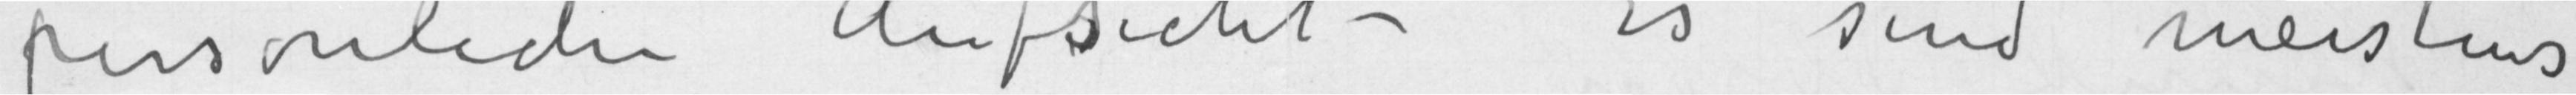personliche Aufsicht – Es sind meistens
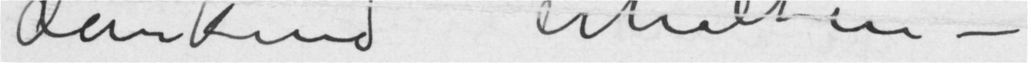dankend erhalten –
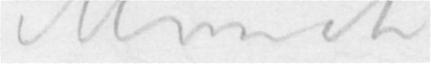Munch
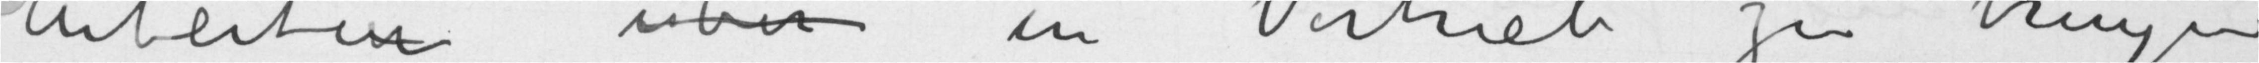Arbeitern uber in Vertrieb zu bringen
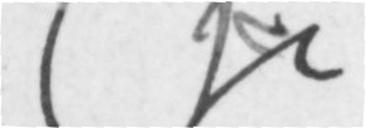(je
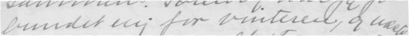bundet mig for vinteren, og næste
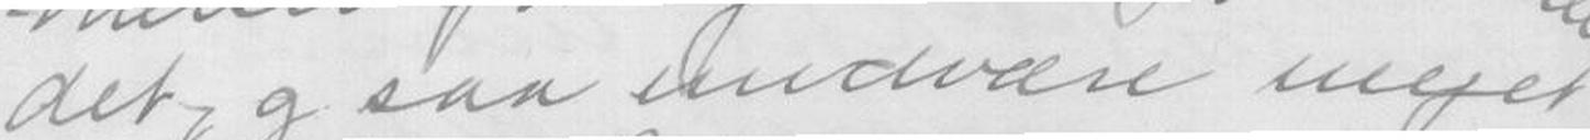det, og saa undvære meget
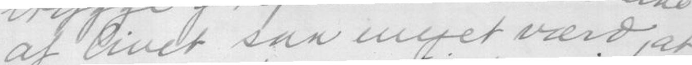af livet saa meget værd, at
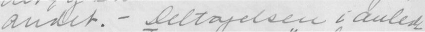andet. - Deltagelsen i anled-
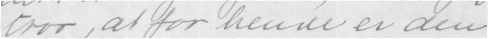tror, at for hende er den
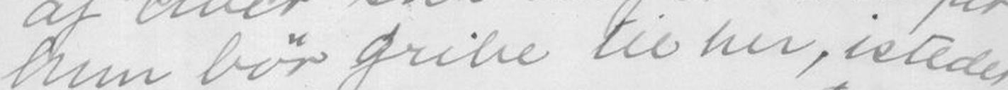hun bør gribe til her, istedet
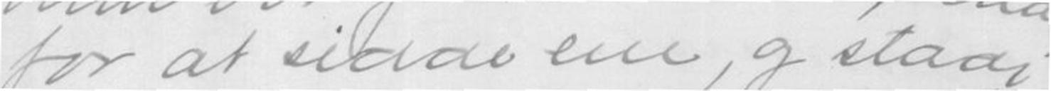for at sidde ene, og staa i
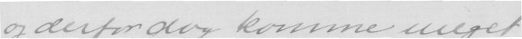og derfor dog komme meget
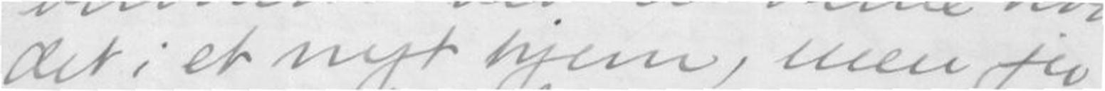det i et nyt hjem, men jeg
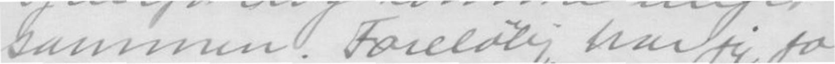sammen. Foreløbig har jeg jo
